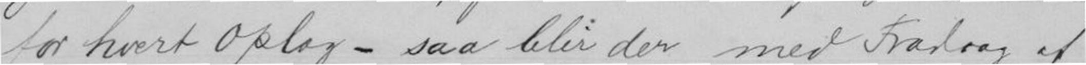for hvert Oplag - saa blir der med Fradrag af
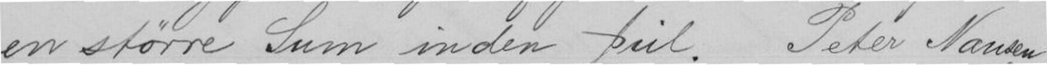en større Sum inden Jul. Peter Nansen
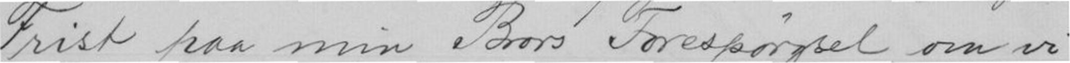Frist paa min Brors Forespørgsel, om vi
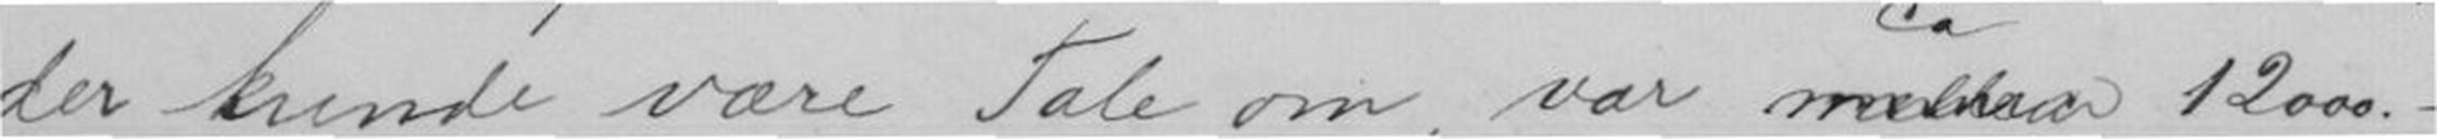der kunde være tale om, var ca 12000.-
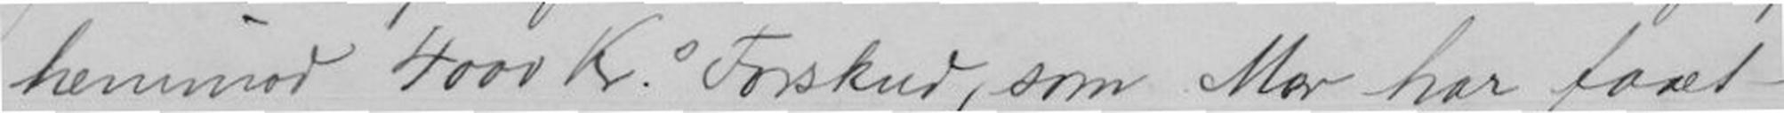henimod 4000 kr. Forskud, som Mor har faaet
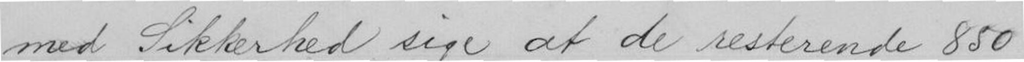med Sikkerhed sige at de resterende 850
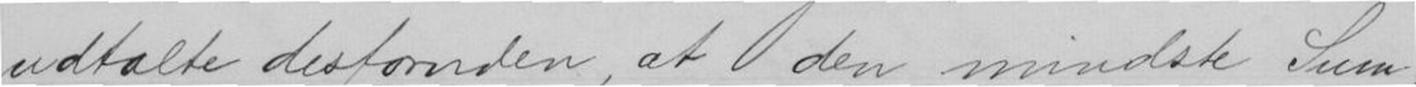udtalte desforuden, at den mindste Sum,
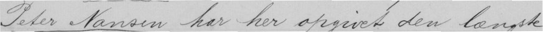Peter Nansen har her opgivet den længste
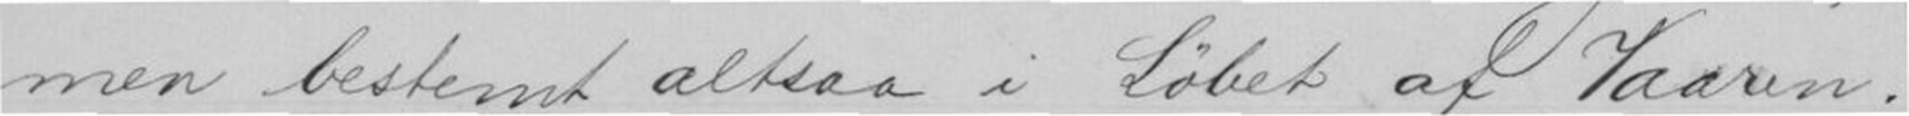men bestemt altsaa i Løbet af Vaaren.
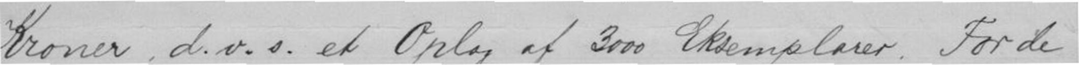Kroner, d.v.s. et Oplag af 3000 Eksemplarer. For de
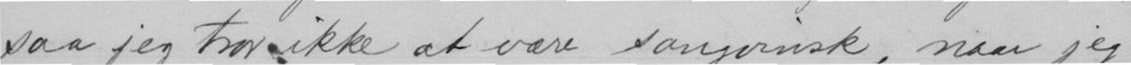saa jeg tror ikke at være sangvinsk, naar jeg
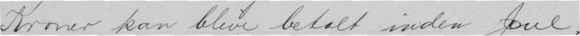Kroner kan blive betalt inden Jul,
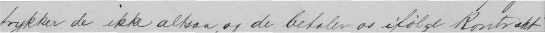trykker de ikke altsaa, og de betaler os ifølge Kontrakt
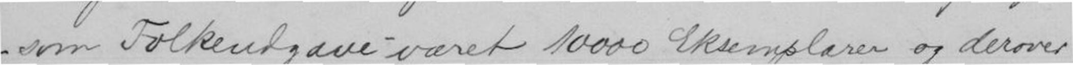- som Folkeudgave været 10000 Eksemplarer og derover,
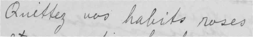Qnittez vos habits roses
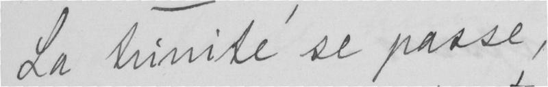La trinite se passe,
